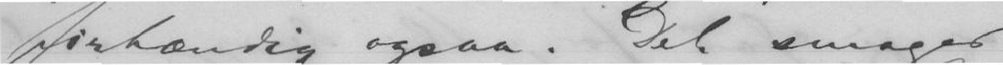firhændig ogsaa. Det smager
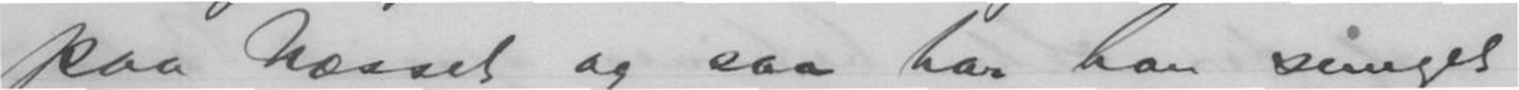paa Næsset og saa har han sunget
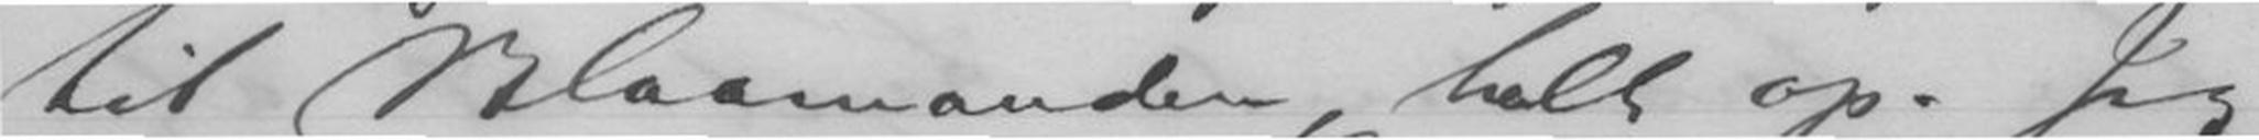gaar jeg Tur til Blaamanden, helt op. Jeg
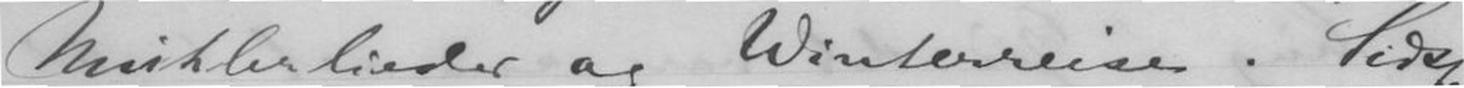Musiklerlieder og Winterreise. Sidste
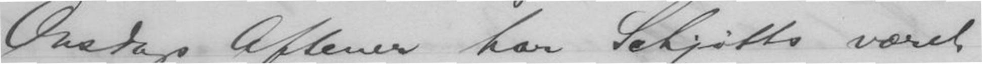Onsdags Aftener har Schjøtts været
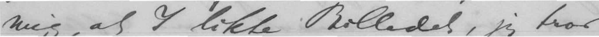mig, at I likte Billedet, jeg tror
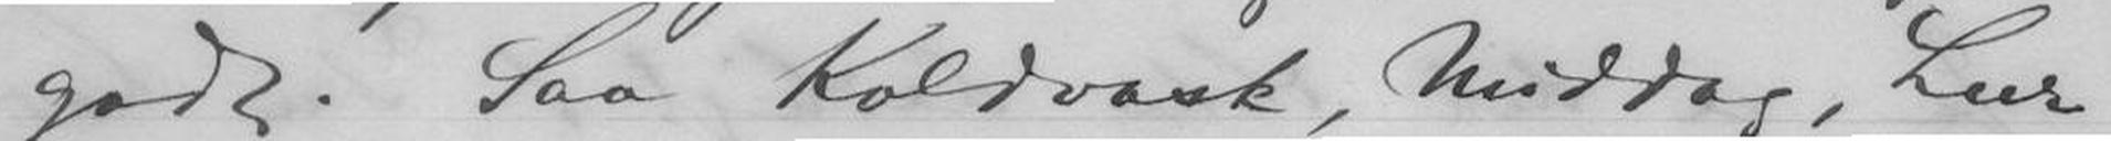godt. Saa Koltvask, Middeg, Lur
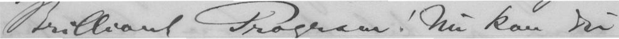Brilliant Program! Nu kan du
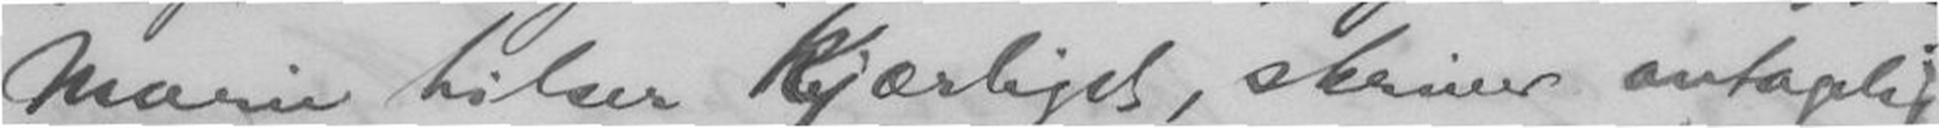Marie hilser kjærligst, skriver antagelig
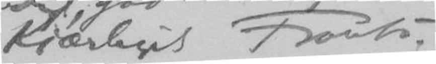Kjærligtst Frants.
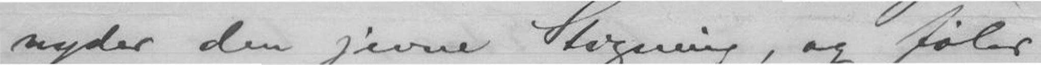nyder den jevne Stigning, og føler
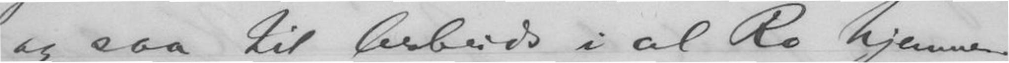og saa til Arbeid i al Ro hjemme
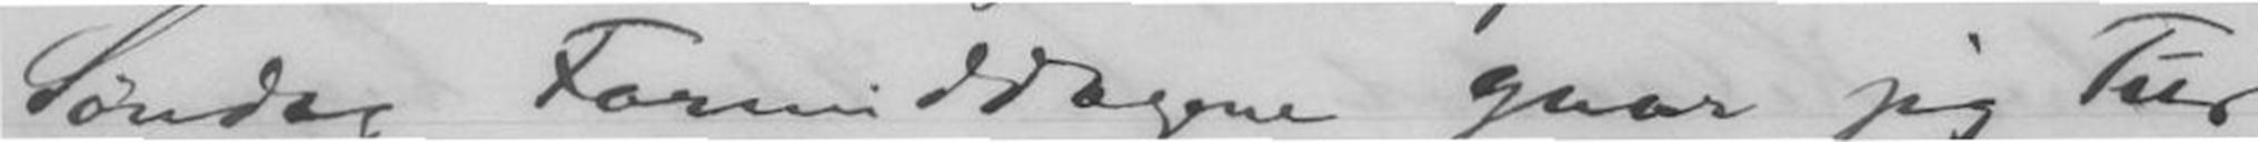Søndag Formiddagene
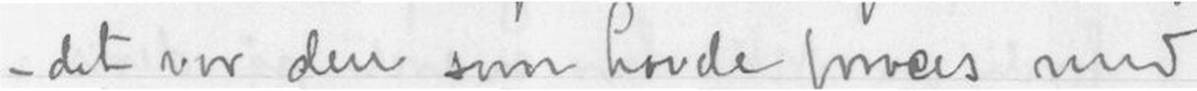det var den som havde proces med
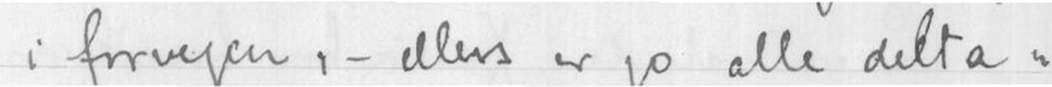i forvejen,- ellers er jo alle delta-
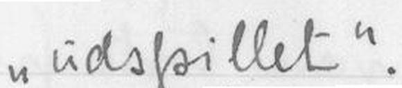udspillet.
